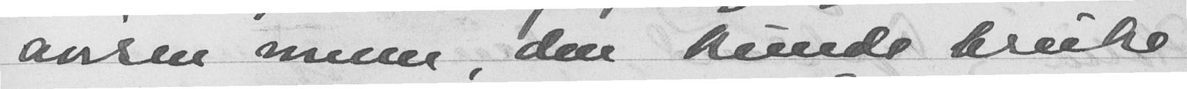avisen mener, den kunde bruke
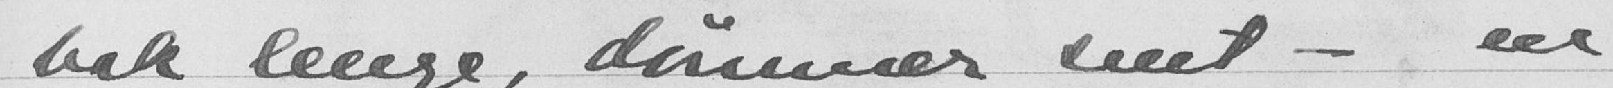bok lenge, dømmer sent - en
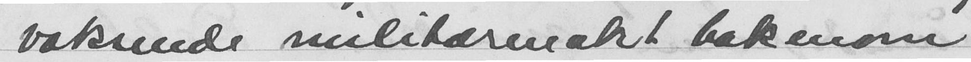voksende militærmakt bakenom
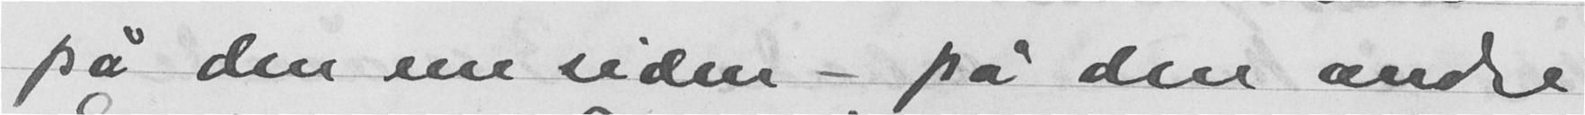på den ene siden - på den andre
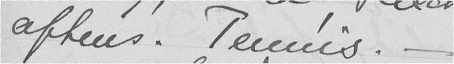aftens. Tennis. -
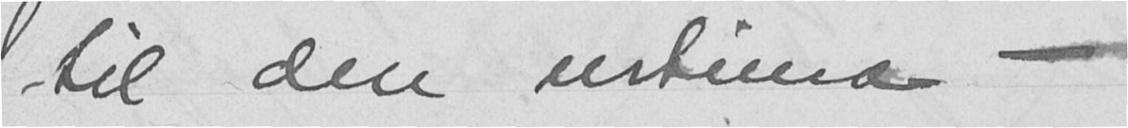til den urtima -
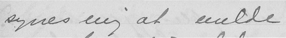synes mig at melde
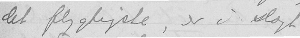det flygtigste, er i slægt
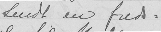Sendt en freds-
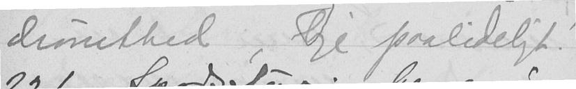drømthed, lige paalideligt!
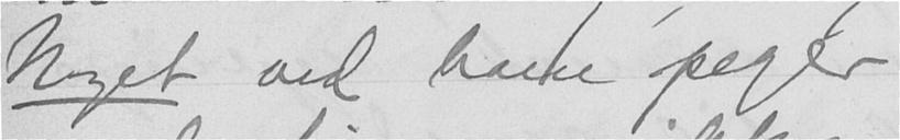Noget ved ham peger
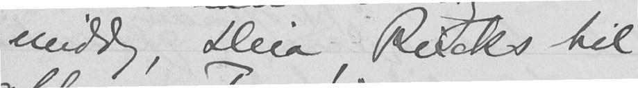middag, Heia, Riecks til
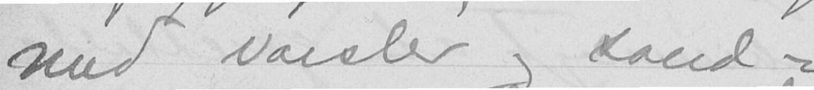med varsler og sand-
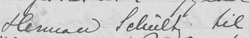Herman Schultz til
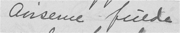Aviserne fulde
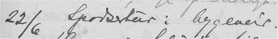22/6 Spadsertur i bygeveir.
